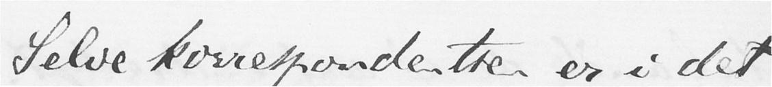Selve korrespondentsen er i det
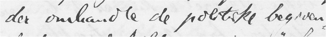der omhandle de politiske begiven-
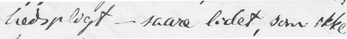hedspligt - saare lidet, som ikke
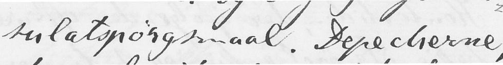sulatspørgsmaal. Depecherne,
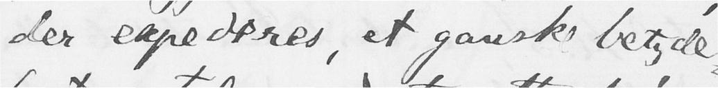der expederes, et ganske betyde-
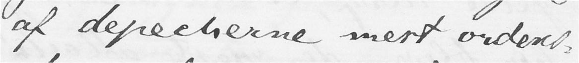af depecherne mest ordens-
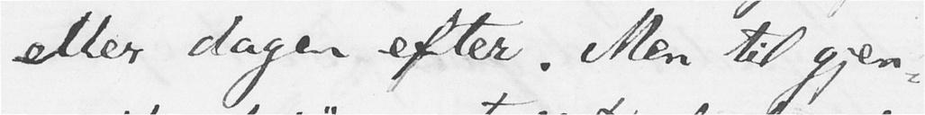eller dagen efter. Men til gjen-
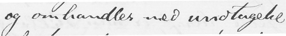og omhandler med undtagelse
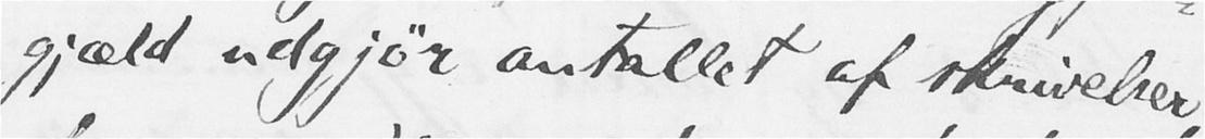gjæld udgjør antallet af skrivelser,
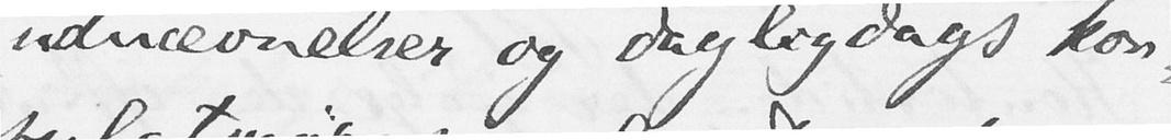udnævnelser og dagligdags kon-
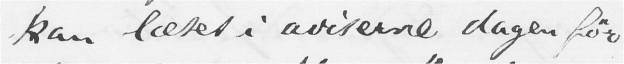kan læses i aviserne dagen før
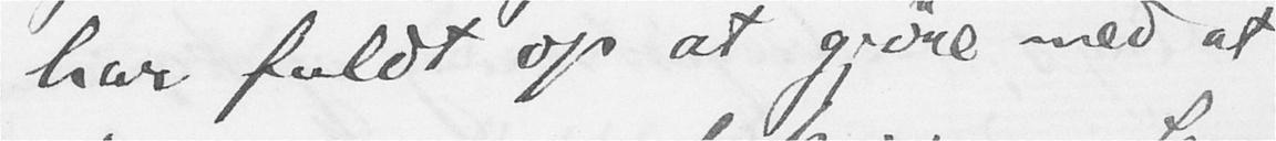har fuldt op at gjøre med at
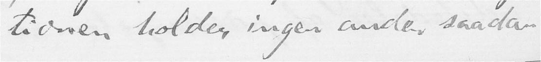tionen holder ingen anden saadan.
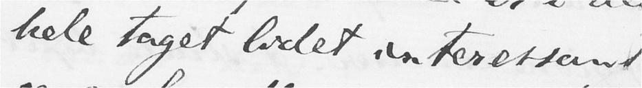hele taget lidet interessant
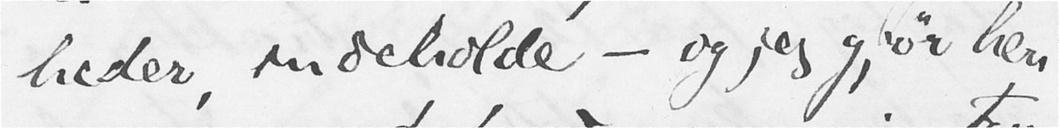heder, indeholde - og jeg gjør her
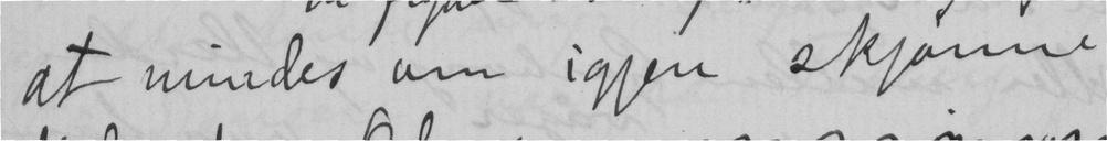at mindes om igjen skjønne
